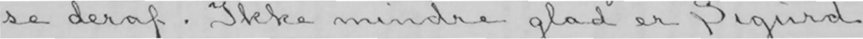se deraf. Ikke mindre glad er Sigurd
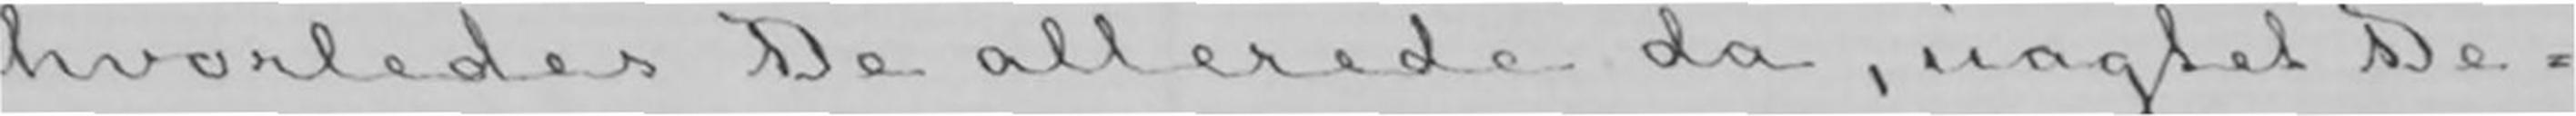hvorledes De allerede da, uagtet De-
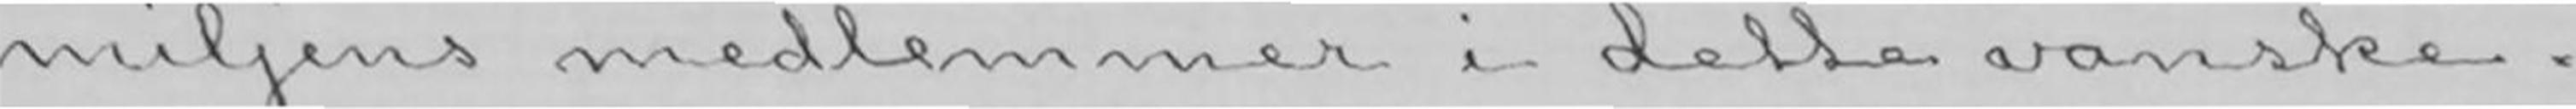miljens medlemmer i dette vanske-
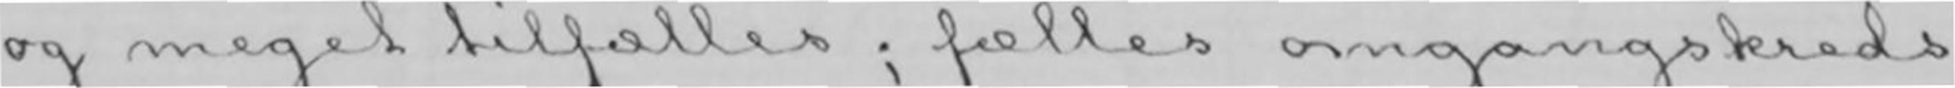og meget tilfælles; fælles omgangskreds
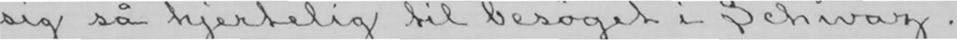sig så hjertelig til besøget i Schwaz.
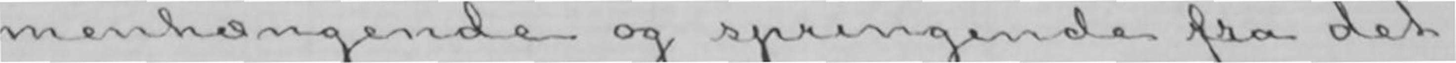menhængende og springende fra det
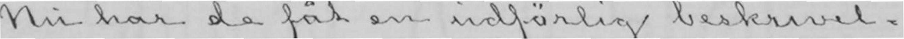Nu har de fåt en udførlig beskrivel-
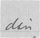din
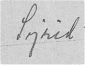Sigrid.
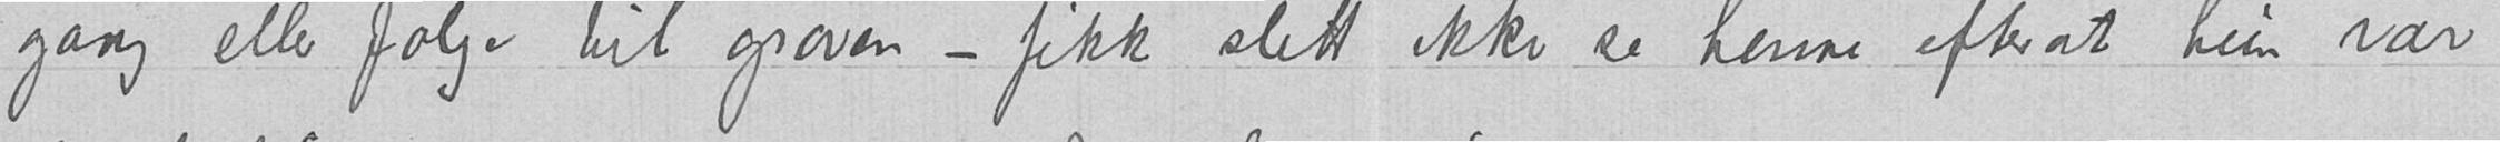gang eller følge til graven – fikk slett ikke se henne efterat hun var
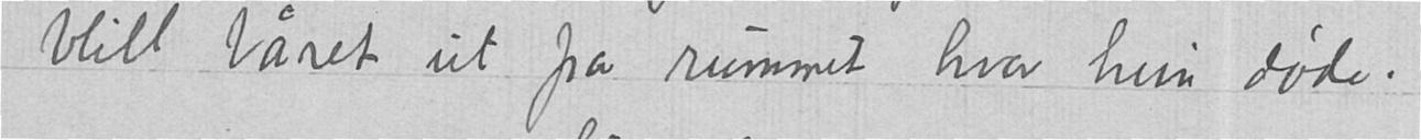blitt båret ut fra rummet hvor hun døde.
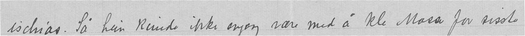ischias. Så hun kunde ikke engang være med å kle Mosse for sisste
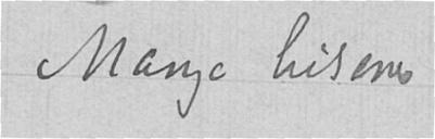Mange hilsener
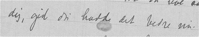dig, gid du hadde det bedre nu.
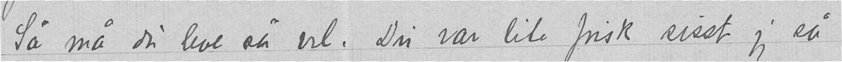Så må du leve så vel. Du var lite frisk sisst jeg så
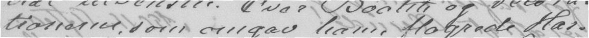tionerne, som omgav ham, flagrede Har-
